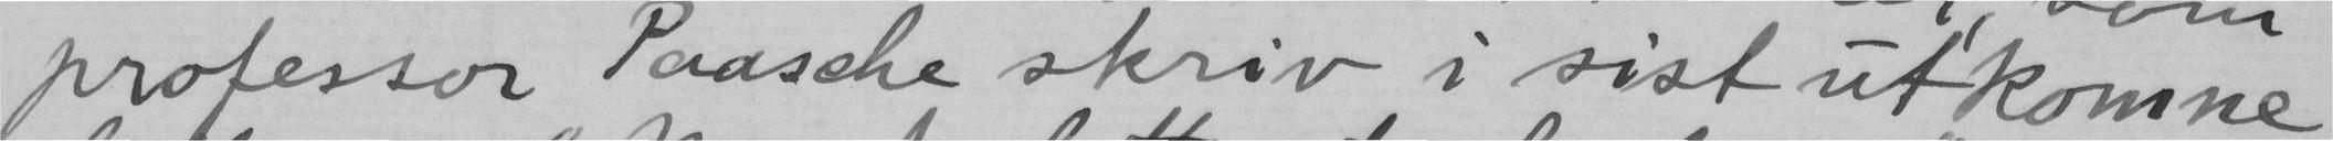professor Paasche skriv i sist utkomne
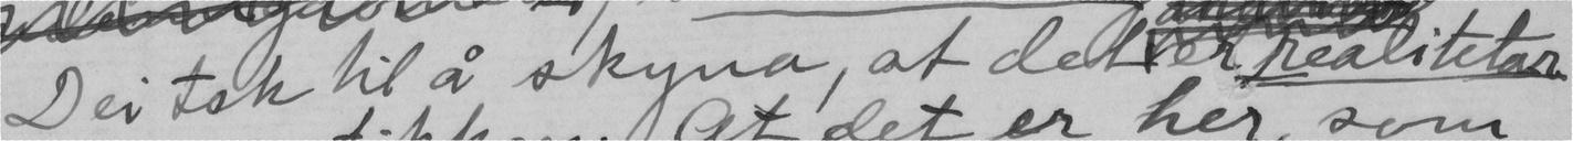Dei tok til å skyna, at det er realitetar
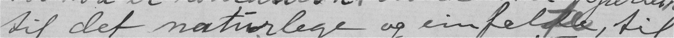til det naturlege og einfelde, til
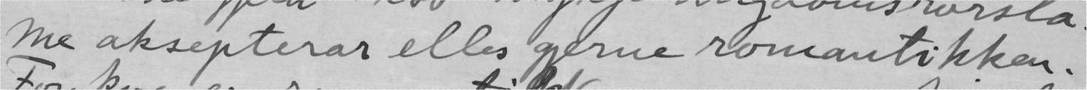Me aksepterar elles gjerne romantikken.
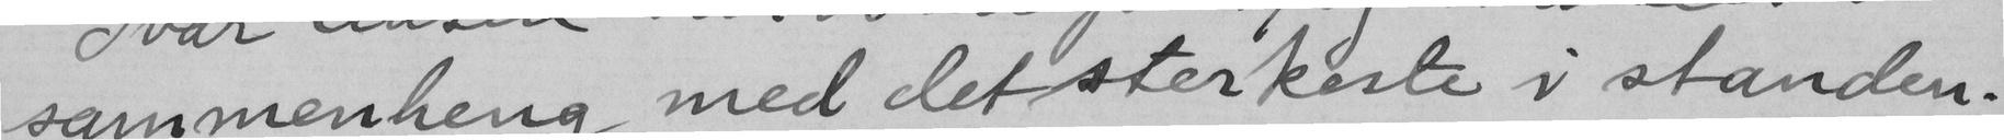sammenheng med det sterkeste i standen.
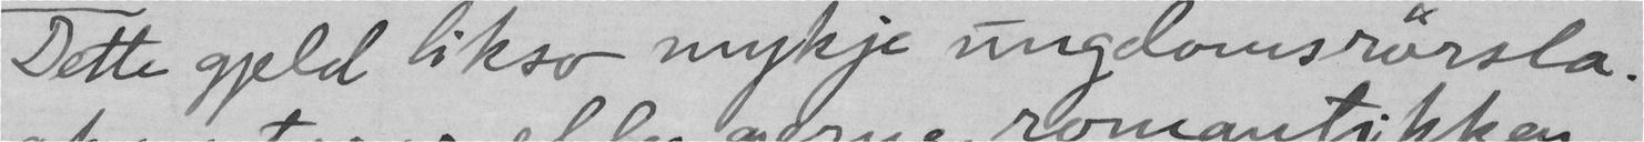Dette gjeld likso mykje ungdomsrørsla.
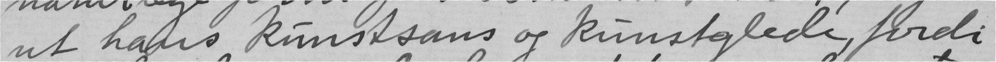ut hans kunstsans og kunstglede, fordi
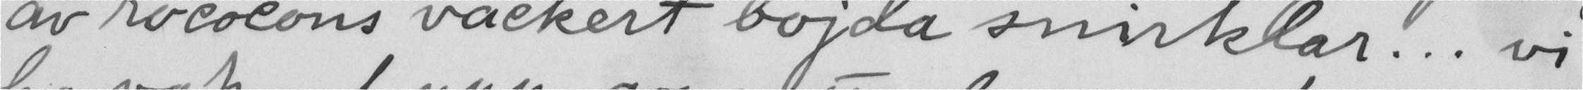av rococons vackert bøjda snirklar... vi
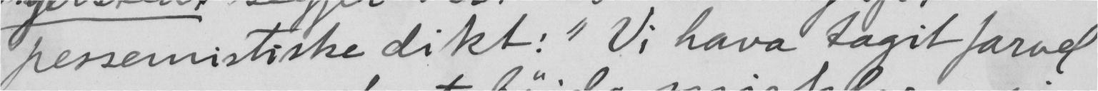pessemistiske dikt: "Vi hava tagit farvel
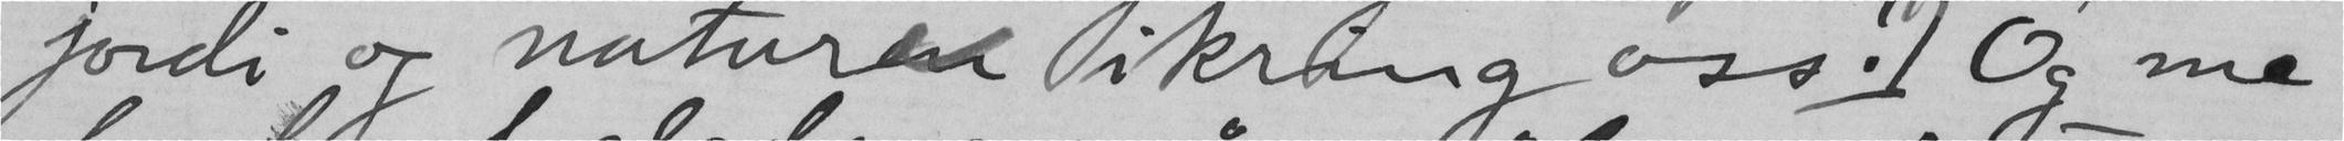jordi og naturen i kring oss! Og me
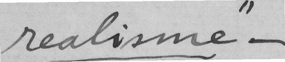realisme" -
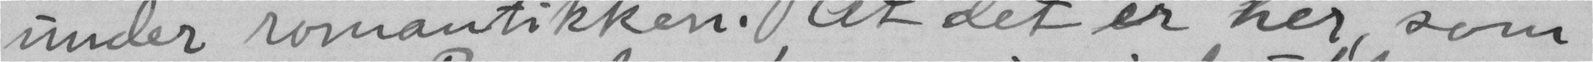under romantikken. At det er her, som
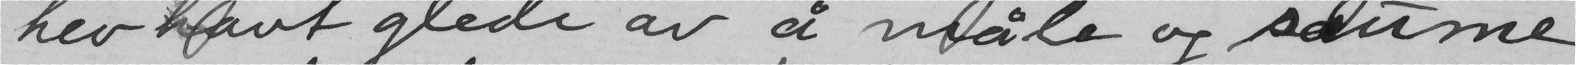hev havt glede av å måle og saume
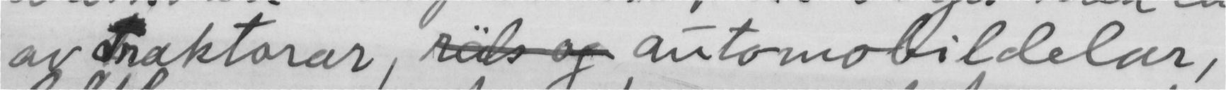av traktorar, räds og automobildelar,
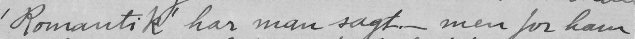'Romantik' har man sagt - men for ham
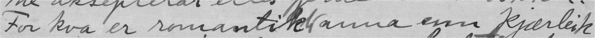For kva er romantik anna enn kjærleik
